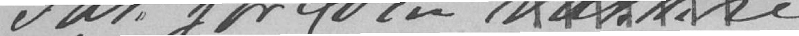Tak for Din vakkre
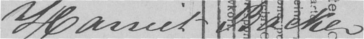Harriet Backer
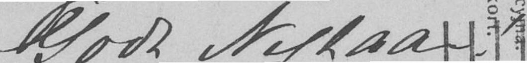Godt Nytaar!
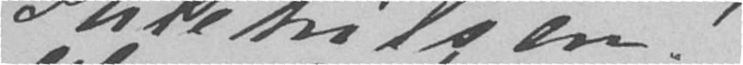Julehilsen!
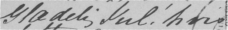Glædelig Jul, hvis
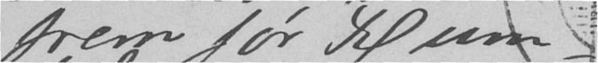frem før Rum-
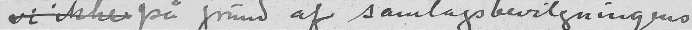vi ikke på grund af samlagsbevilgningens
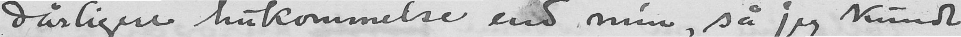dårligere hukommelse end min, så jeg kunde
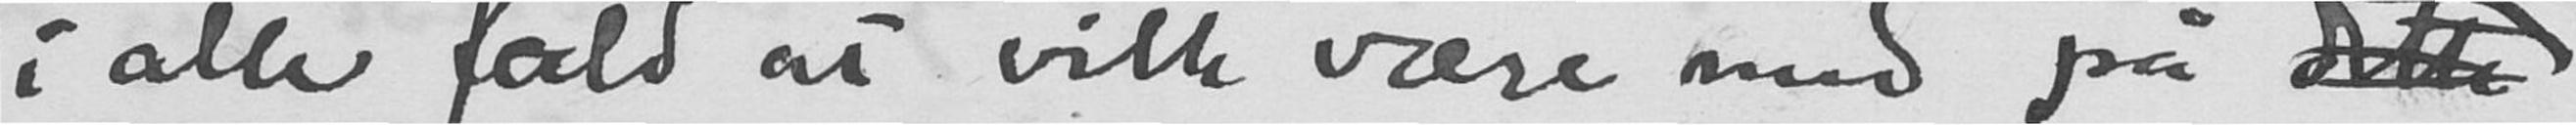i alle fald at ville være med på dette
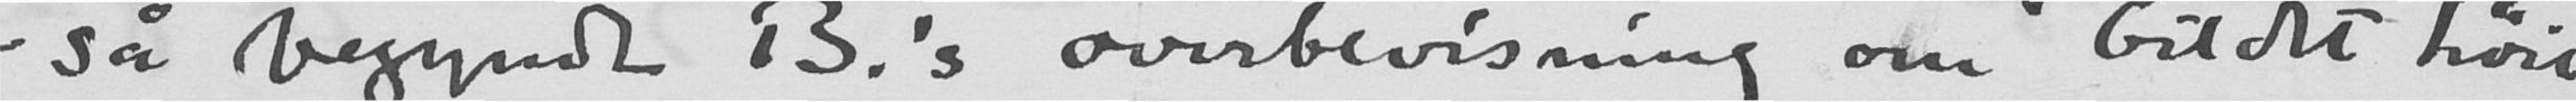- så begyndte B.'s overbevisning om bildets høie
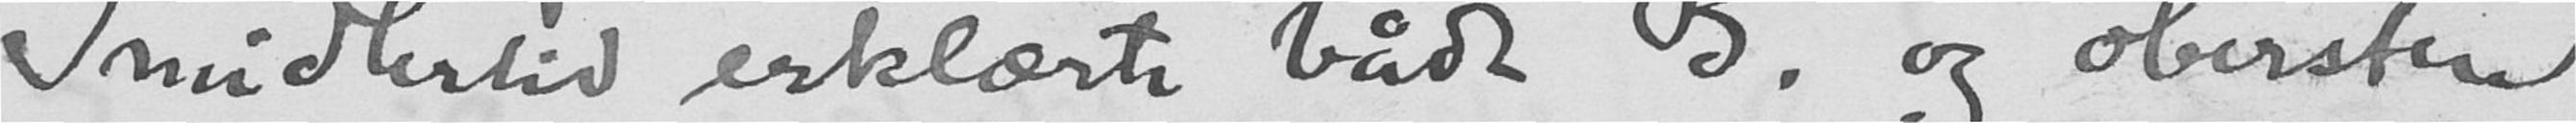Imidlertid erklærte både B. og obersten
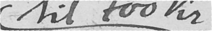til 700 kr.
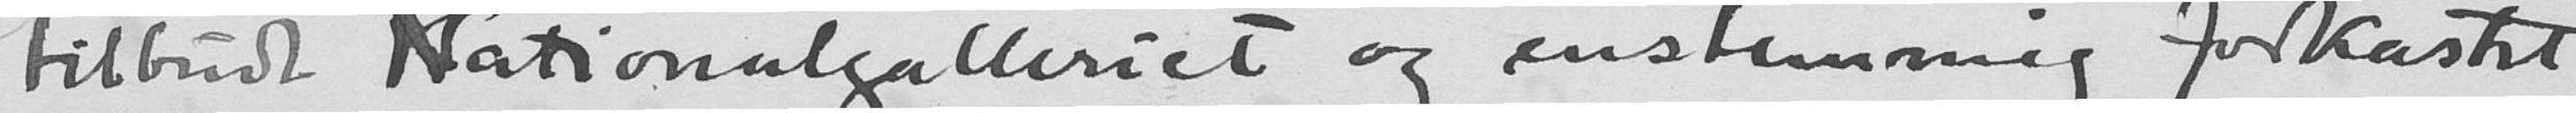tilbudt Nationalgalleriet og enstemmig forkastet
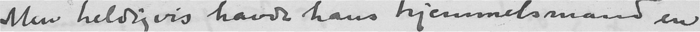Men heldigvis havde hans hjemmelsmand en
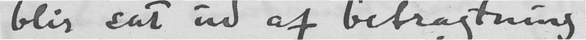- blir sat ud af betragtning).
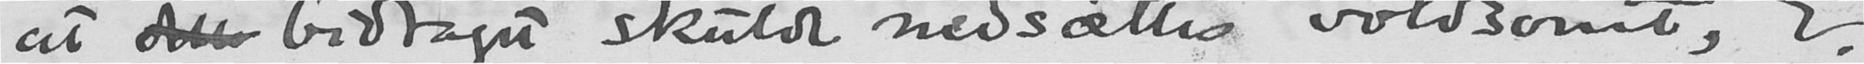at dette bidraget skulde nedsættes voldsomt,
